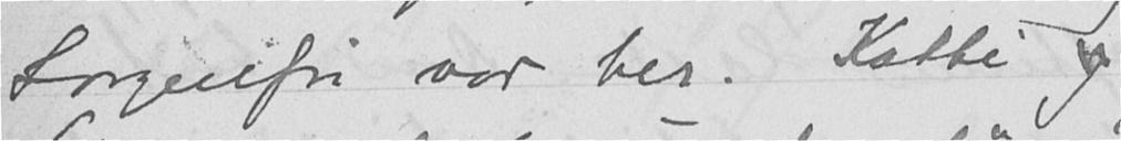Sorgenfri var her. Katti og
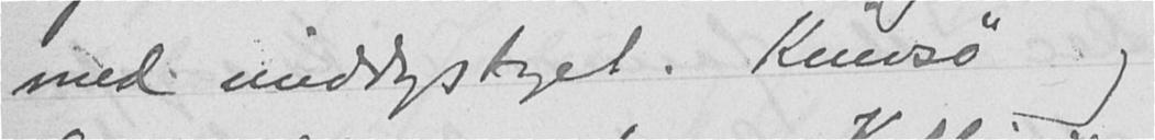med middagstoget. Knivsø og
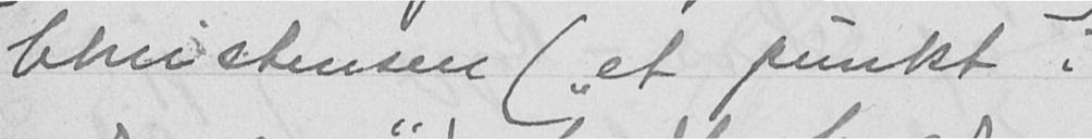Christensen ("et punkt i
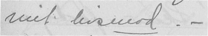mit livsmod. -
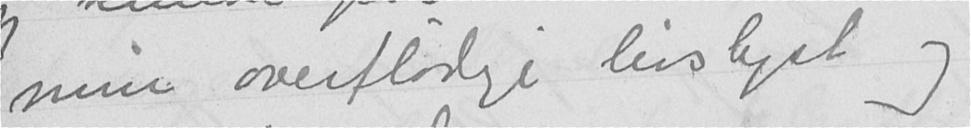min overflødige livslyst og
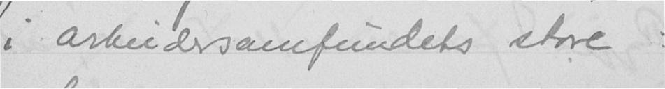i arbeidersamfundets store
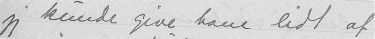jeg kunde give ham lidt af
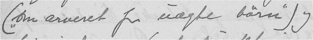("om arveret for uægte børn") og
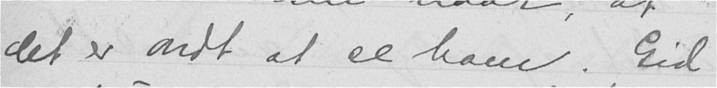det er ondt at se ham. Gid
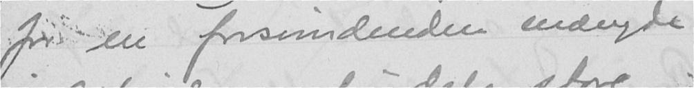for en forsvindende mængde
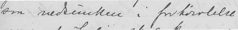saa nedsunken i fortvivlelse
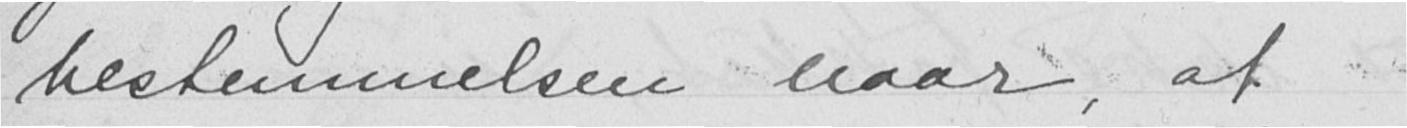bestemmelsen naar, at
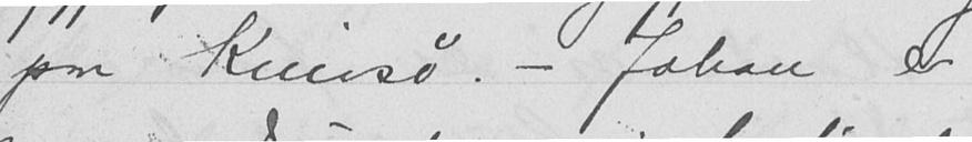paa Knivsø. - Johan er
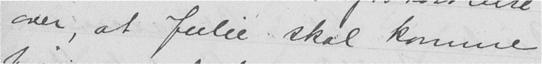over, at Julie skal komme
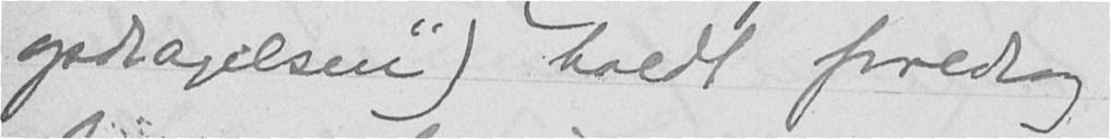opdragelsen") holdt foredrag
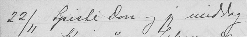22/11 Spiste Don og jeg middag
